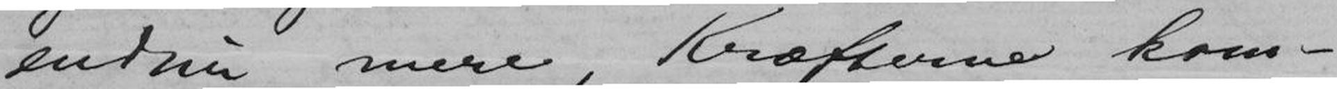endnu mere, Kræfterne kom-
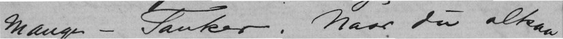Mange - Tanker. Naar du altsaa
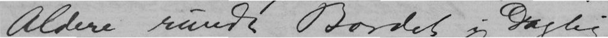Aldere rundt Bordet i Daglig-
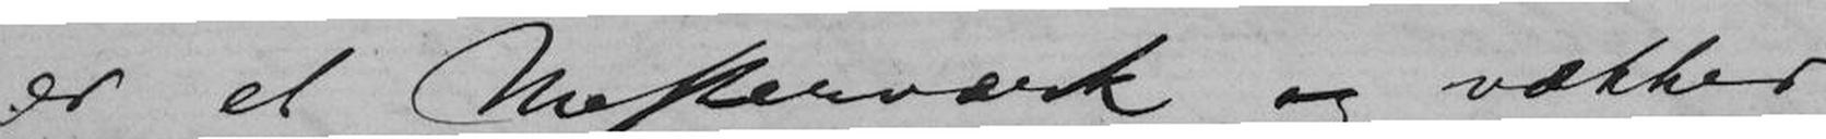er et Mesterværk og vakker
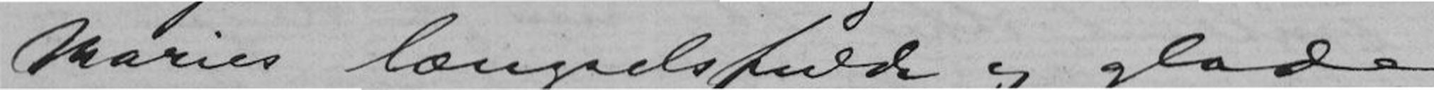Maries længselsfulde og glade
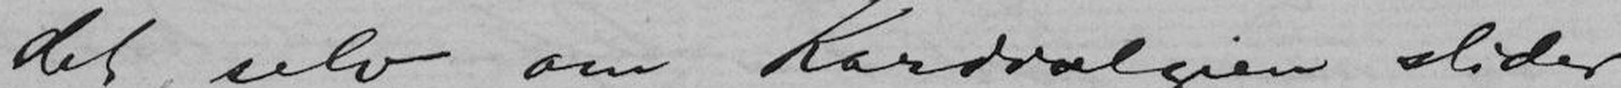det, selv om Kardialgien slider
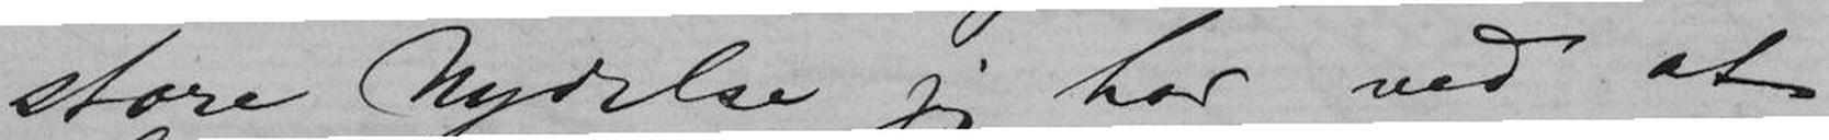store Nydelse jeg har ved at
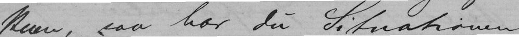stuen, saa har du Situationen
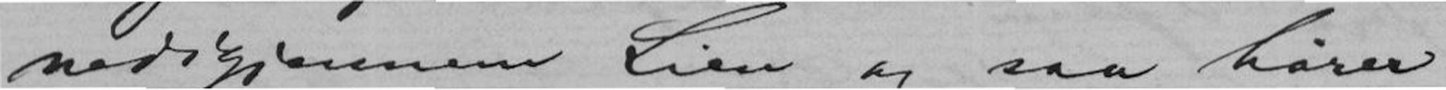nedigjennem Lien og saa hører
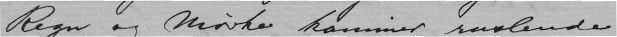Regn og Mørke kommer ruslende
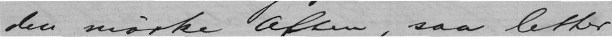den mørke Aften, saa letter
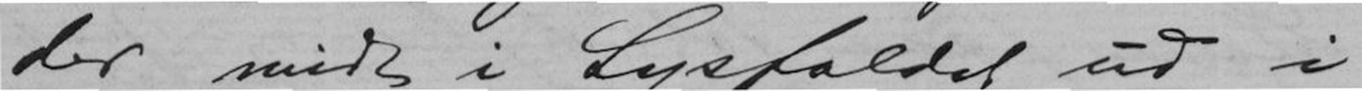der midt i Lysfaldet ud i
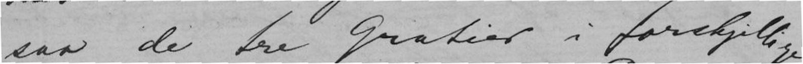saa de tre Gratier i forskjillige
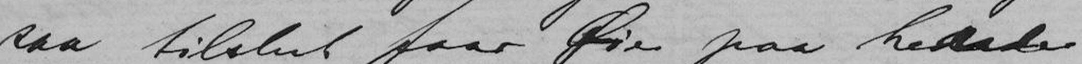saa tilslut faar Øie paa hende
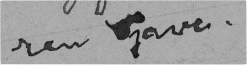ren Gave.
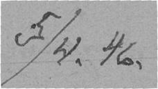5/4. 46.
